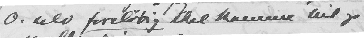O. selv foreløbig skal komme hit op
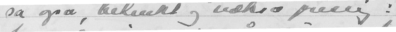sa også, betenkt og nokså pussig:
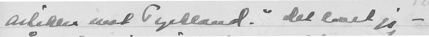Artikler mot Tyskland." Det lovet jeg -
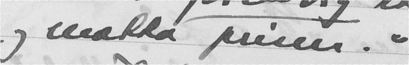og motta prisen."
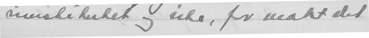mustetutet og rike, for makt det
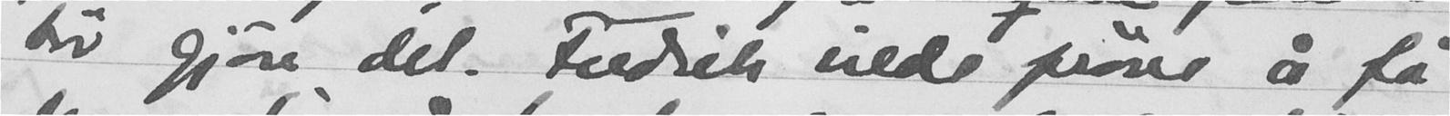tør gjøre det. Fredrik vilde prøve å få
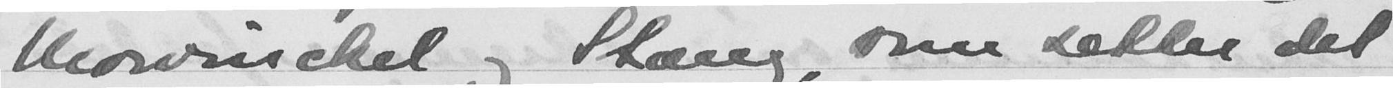Mowinckel og Stang, som setter det
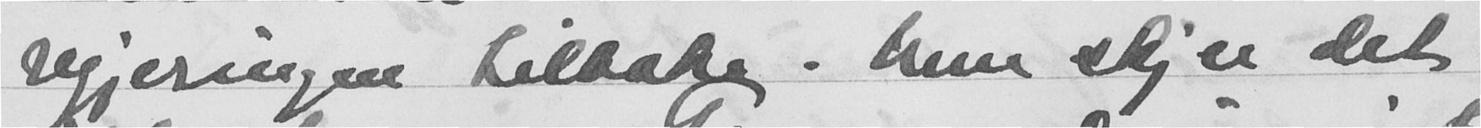regjeringen tilbake. Men skjer det
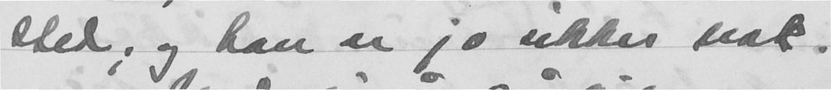sted, og han er jo sikker nok.
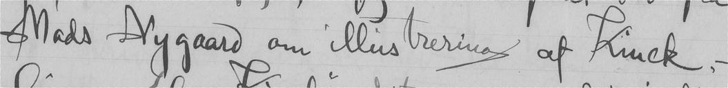Mads Nygaard om illustrering af Kinck, -
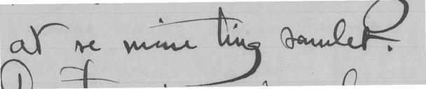at se mine ting samlet.
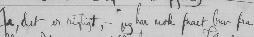Ja, det er rigtigt, - jeg har nok faaet brev fra
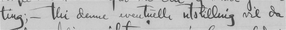ting; - thi denne eventuelle utstilling vil da
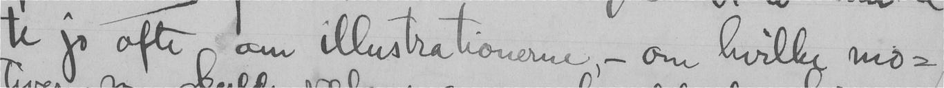te jo ofte, om illustrationerne, - om hvilke mo-
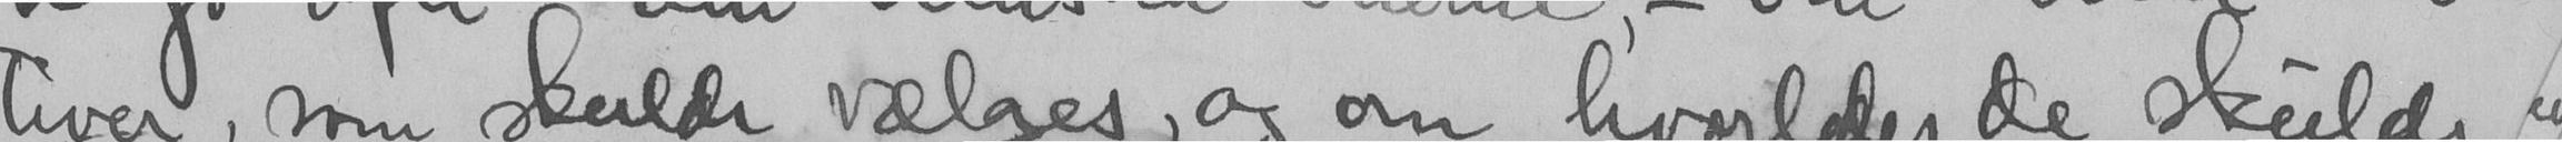tiver, som skulde vælges, og om hvorledes de skulde
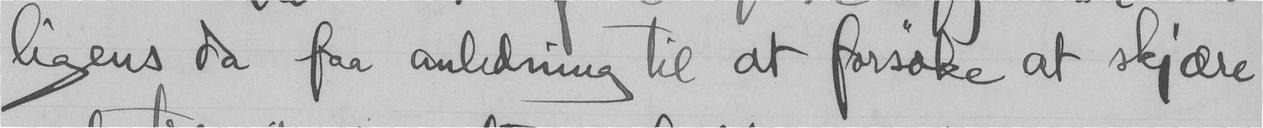ligens da faa anledning til at forsøke at skjære
